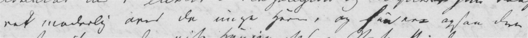ret moderlig over de unge Herrer, og hun ere ogsaa den
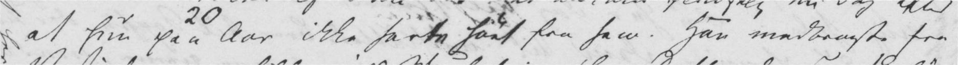at hun paa Aar ikke havde hørt fra ham. Han medbragte fra
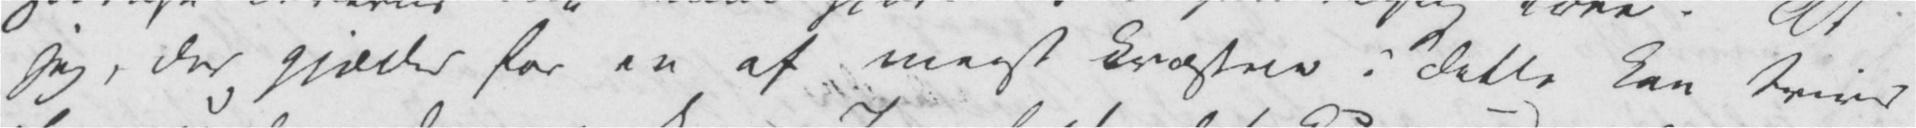jeg, der gjælder for en af meest Kræssne i dette kan trives
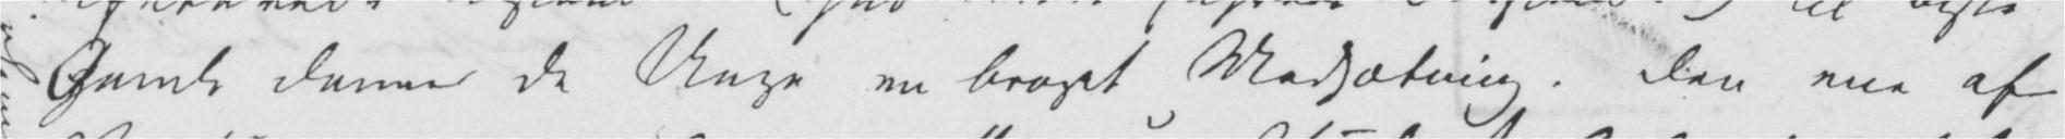Gamle danne de Unge en broget Modsætning. Den ene af
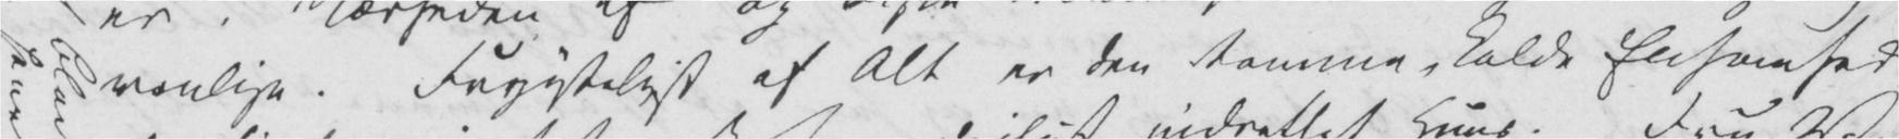venlige. Frygteligst af Alt er den tomme, kolde Ensomhed,
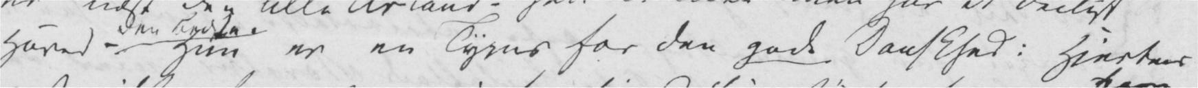Hoved –  Hun er en Typus for den gode Danskhed: Hjertens
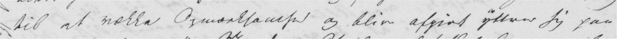til at vække Opmærksomhed og blive afgjort yttrer sig paa
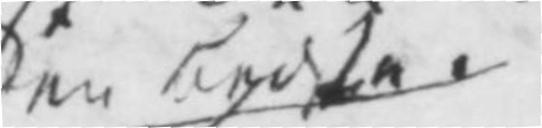Den Bedste.
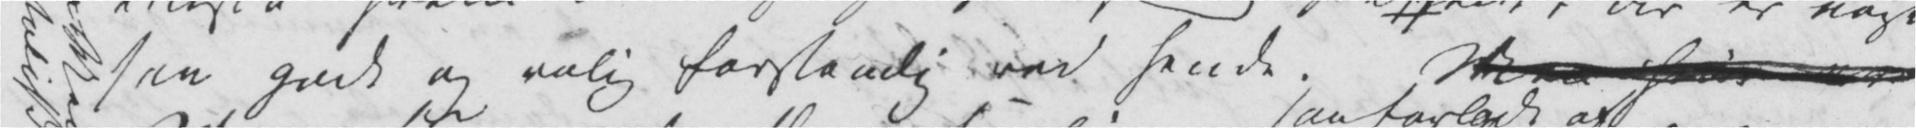saa godt og rolig forstandig ved hende. Men hun er
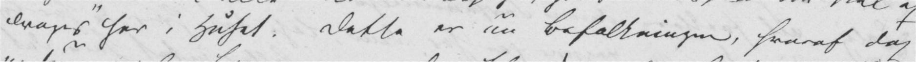drages her i Huset. Dette er nu Befolkningen, hvoraf dog
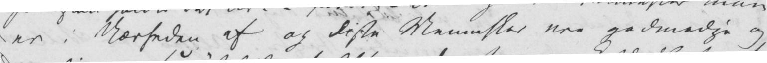i Nærheden af og disse Mennesker ere godmodige og
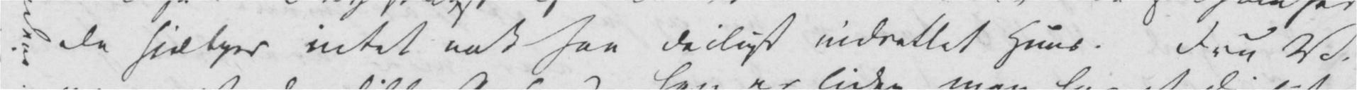da hjælper intet nok saa deiligt indrettet Huus. Fru V.
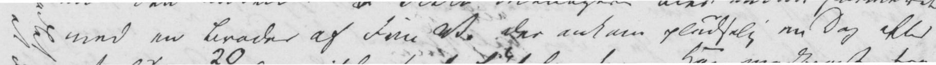med en Broder af Fru V. der ankom pludselig en Dag efter
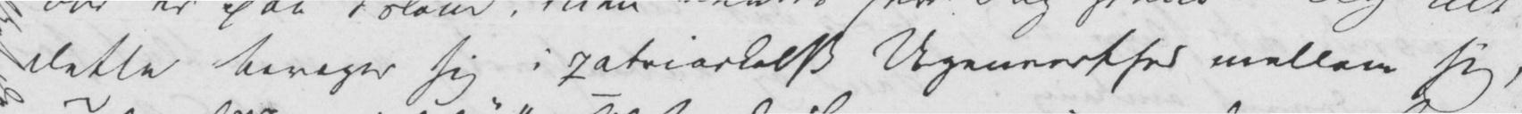dette bevæger sig i patriarkalsk Ugenerthed mellem sig,
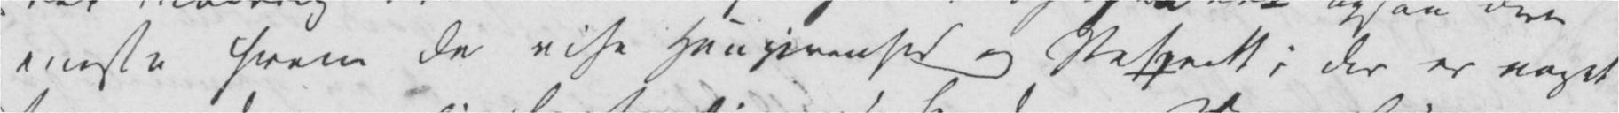eneste hvem de vise Hengivenhed og Respeckt; der er noget
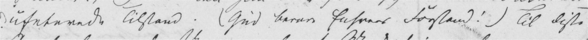ufeterede Tilstand. (Gud bevare Enhvers Forstand!) Til disse
